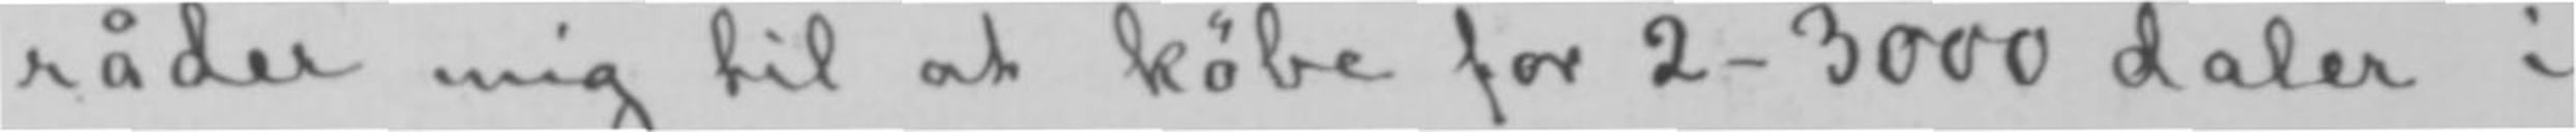råder mig til at købe for 2-3000 daler i
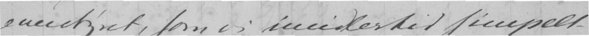eventyret, som vi imidlertid simpelt-
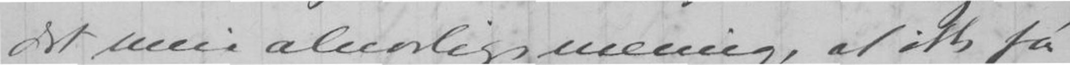det min alvorlige mening, at ikke før
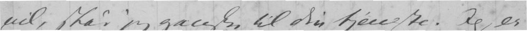vil, står jeg ganske til din tjeneste. Og er
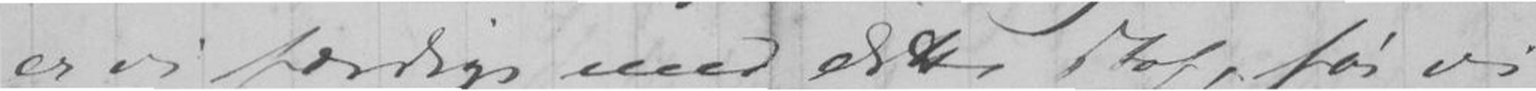er vi færdige med dette stof, før vi
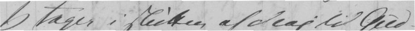Jeg tager i slutten af Maj til Gud-
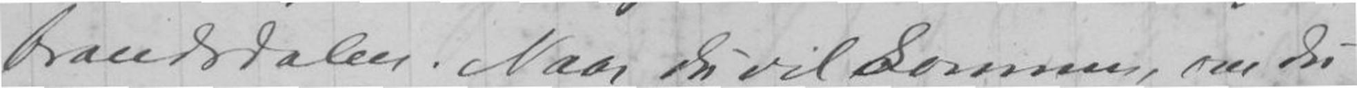brandsdalen. Naar du vil komme, om du
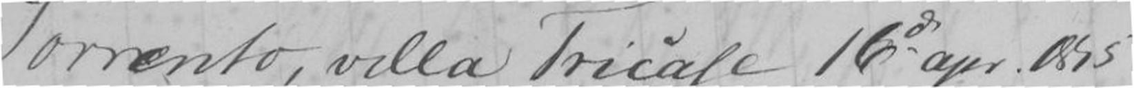Sorrento, villa Tricase 16de apr. 1895
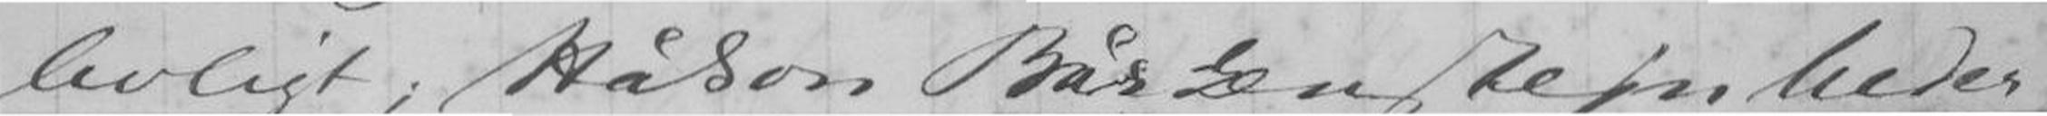livligt, Håkon Bårzenstejn heder
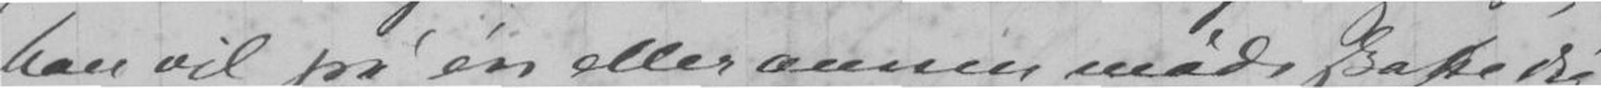han vil på en eller annen måde skaffe dig
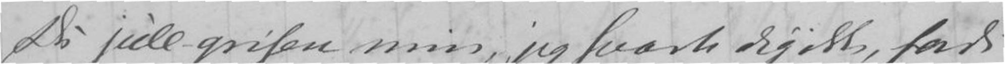Du jule-grisen min, jeg svarte dig ikke, fordi
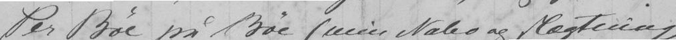Per Bøe på Bøe (min Nabo og slægtning)
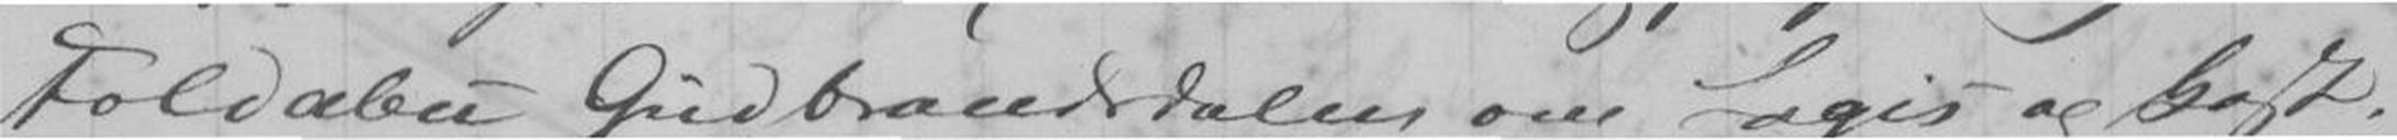Foldabu Gudbrandsdalen om Logis og kost,
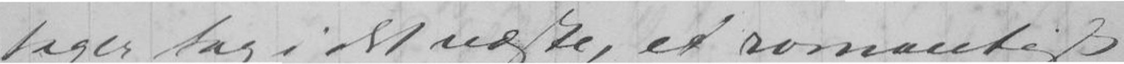tager tag i det næste, et romantisk
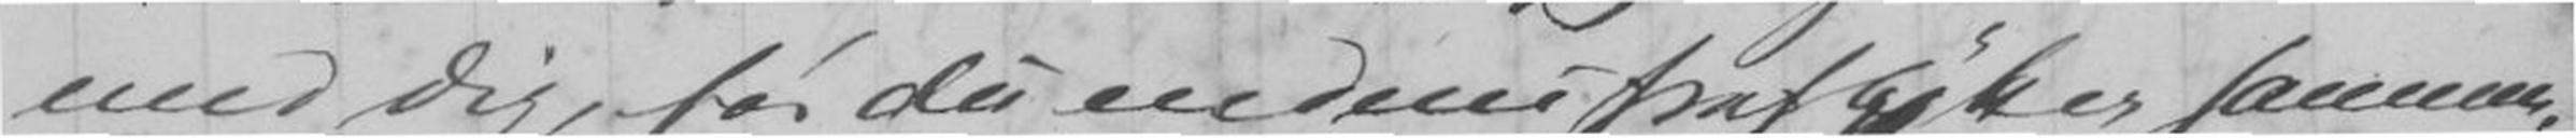med dig, før du endnu fraflytter sammen.
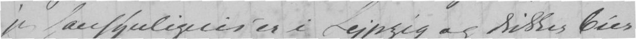jeg sansynligvis er i Leipzig og drikker bier
